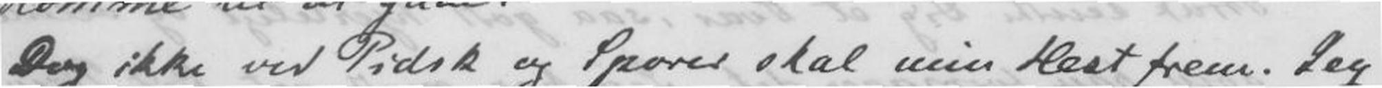Dog ikke ved Pidsk og Sporer skal min Hest frem. Jeg
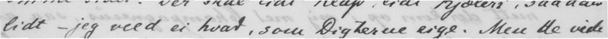lidt - jeg veed ei hvad, som Digterne eige. Men De vide
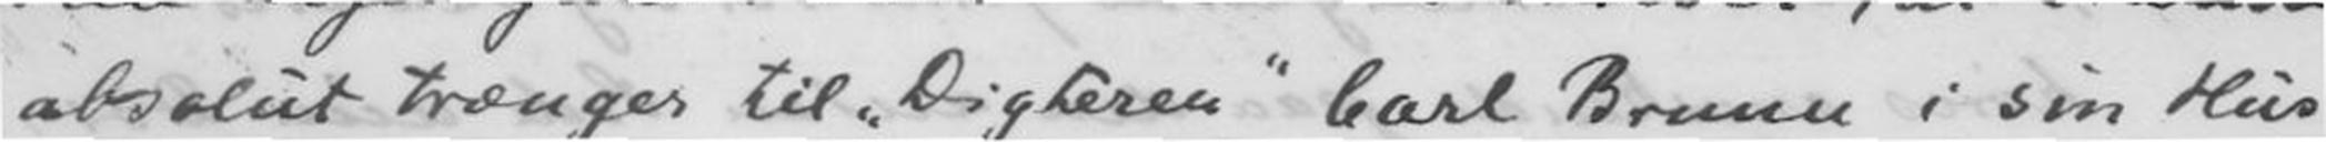absolut trænger til Digteren Carl Bruun i sin Hus-
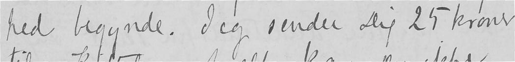hed begynde. Jeg sender Dig 25 kroner
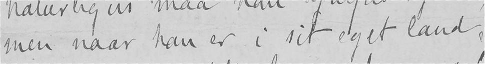men naar han er i sit eget land,
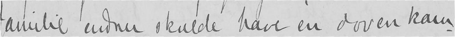familie endnu skulde have en doven kam-
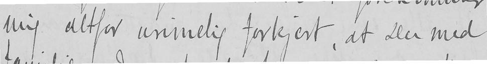mig altfor urimelig forkjert, at Du med
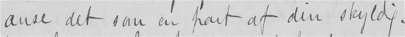anse det som en part af din skyldig-
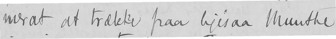merat at trække paa ligesaa Munthe
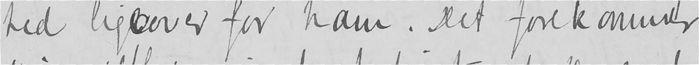hed ligeover for ham. Det forekommer
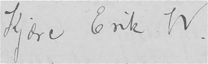Kjære Erik W.
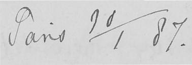Paris 10/1 87.
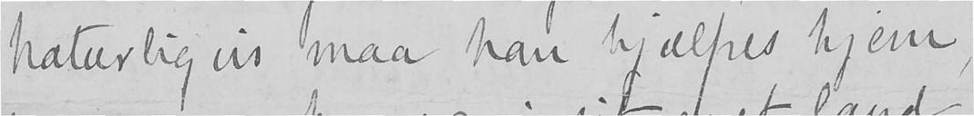Naturligvis maa han hjælpes hjem,
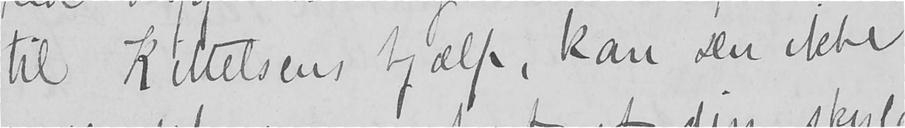til Kittelsens hjælp, kan Du ikke
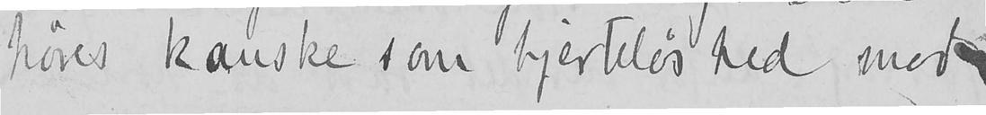høres kanske som hjerteløshed mod
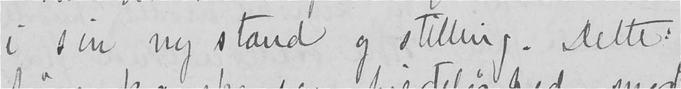i sin ny stand og stilling. Dette
